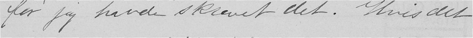før jeg havde skrevet det. Hvis det
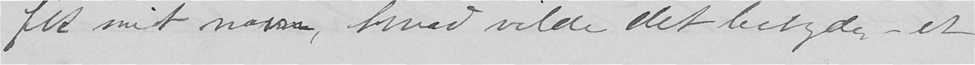fik mit navn, hvad vilde det betyde - et
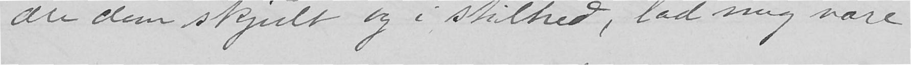ære dem skjult og i stilhed, lad mig være
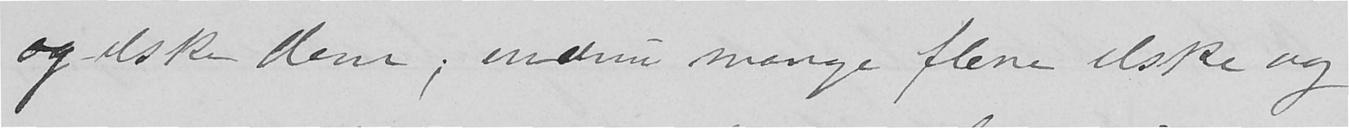og elske dem; endnu mange flere elske og
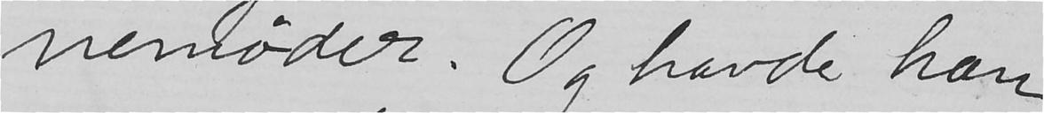nemøder. Og havde han
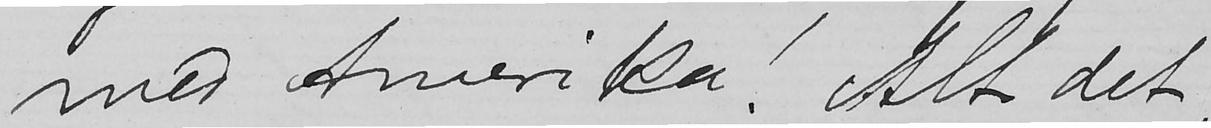med Amerika! Alt det,
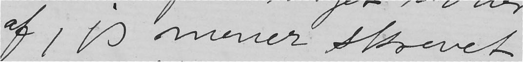af, jeg mener skrevet
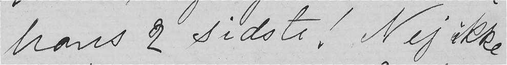hans 2 sidste! Nej ikke
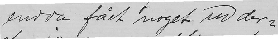endda fået noget ud der-
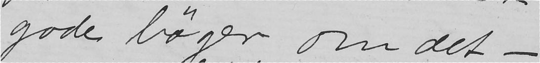gode bøger om det -
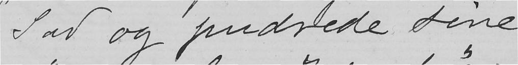Sad og pudrede sine
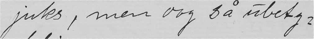juks, men dog så ubety-
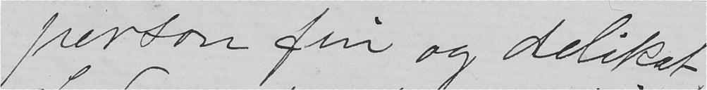person fin og delikat.
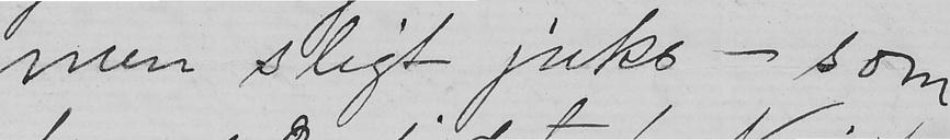men sligt juks - som
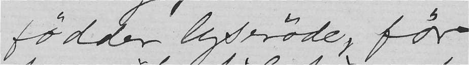fødder lyserøde, før
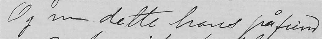Og nu dette hans påfund
